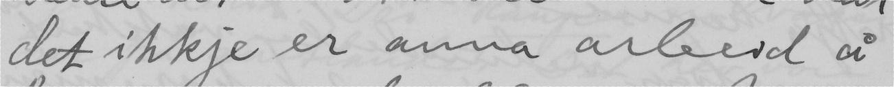det ikkje er anna arbeid å
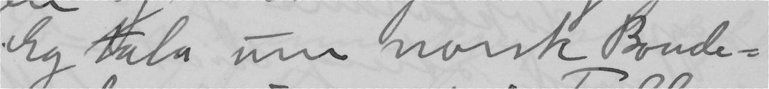Eg tala um norsk Bonde-
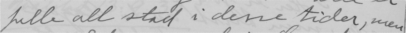fulle all stad i desse tider, men
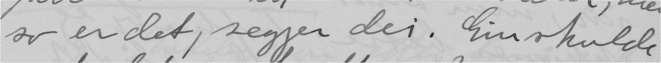so er det, segjer dei. Ein skulde
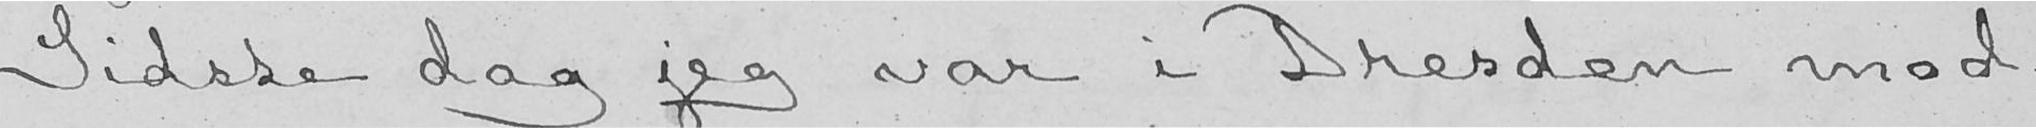Sidste dag jeg var i Dresden mod-
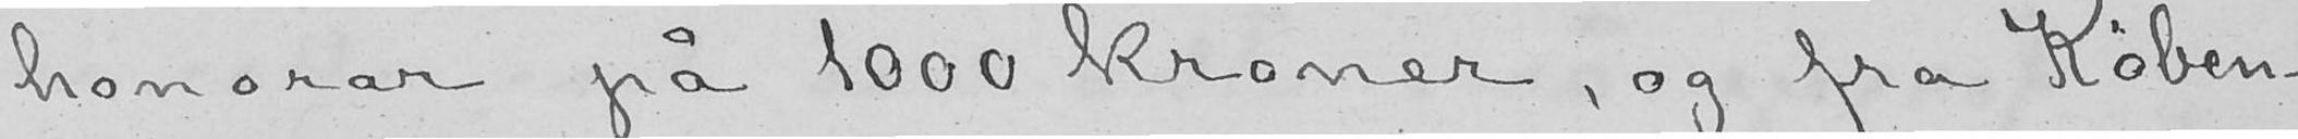honorar på 1000 kroner, og fra Køben-
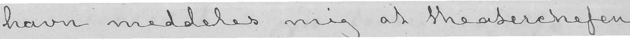havn meddeles mig at theaterchefen
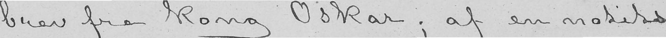brev fra kong Oskar; af en notits
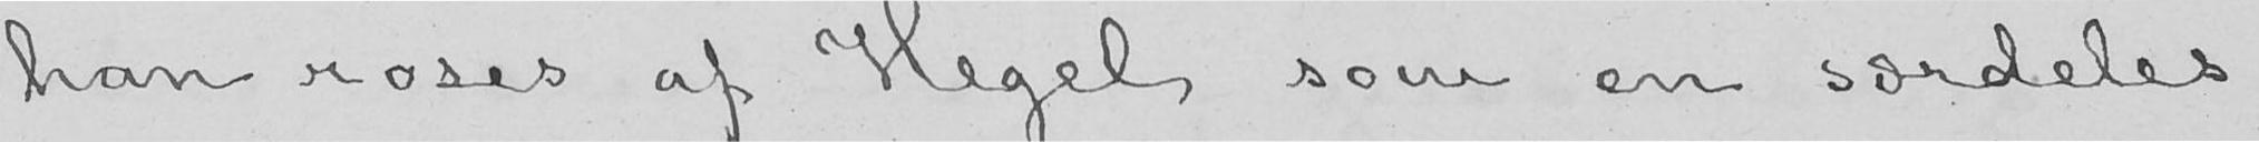han roses af Hegel som en særdeles
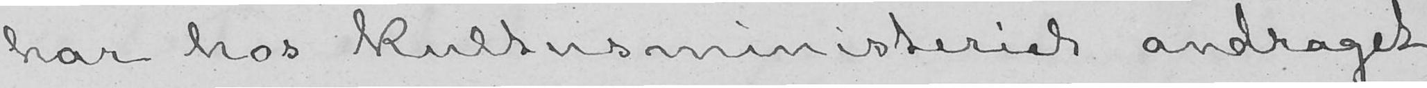har hos kultusministeriet andraget
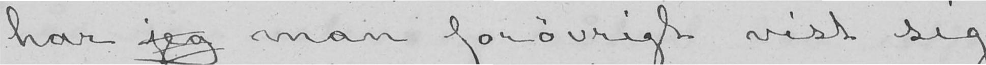har jeg man forøvrigt vist sig
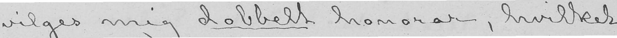vilges mig dobbelt honorar, hvilket
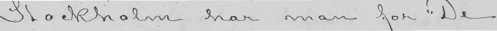Stockholm har man for «De
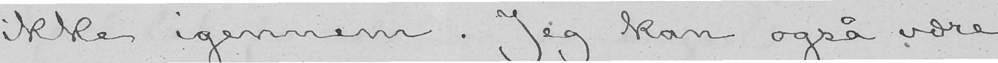ikke igennem. Jeg kan også være
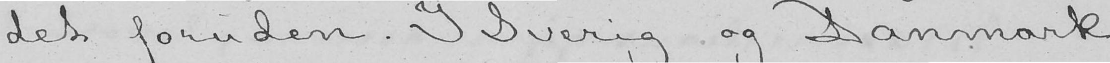det foruden. I Sverig og Danmark
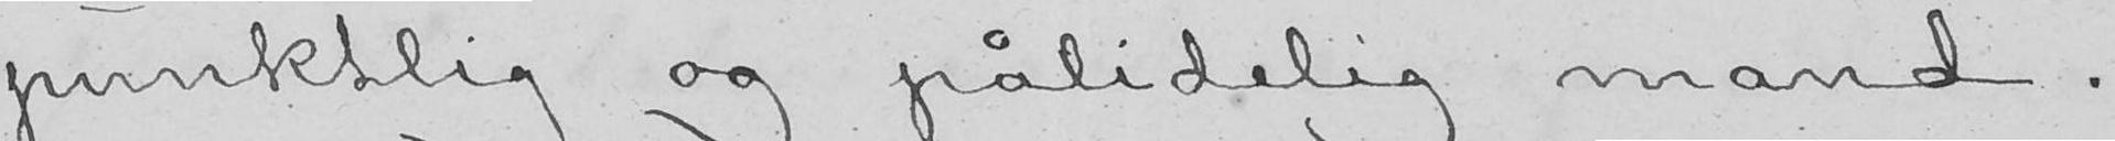punktlig og pålidelig mand.
